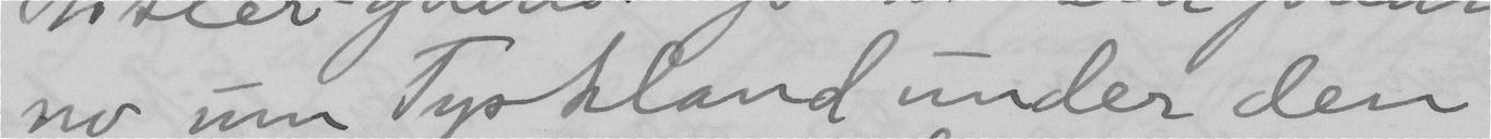no um Tyskland under den
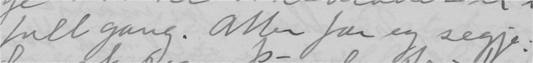full gang. Atter far eg segje:
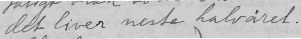det liver neste halvåret.
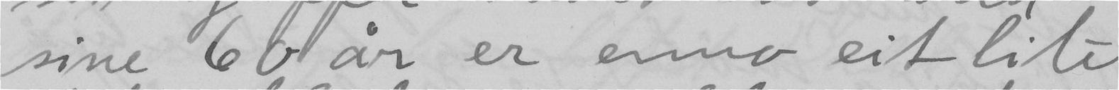sine 60 år er enno eit lite
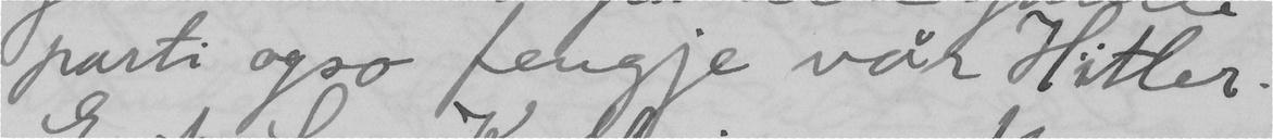parti ogso fengje vår Hitler.
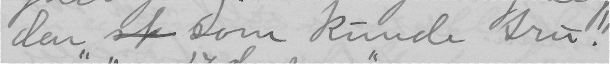den  som kunde tru!
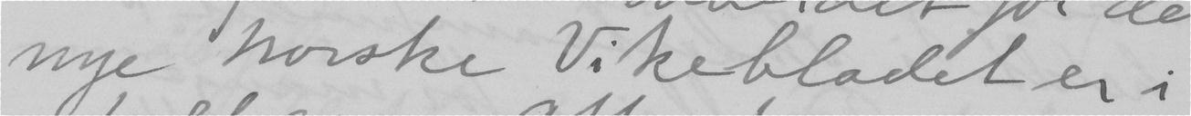nye norske Vikebladet er i
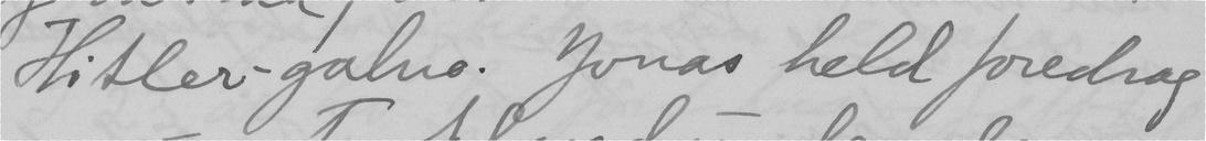Hitlergalne. Jonas held foredrag
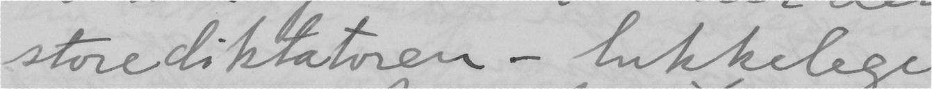store diktatoren – lukkelege
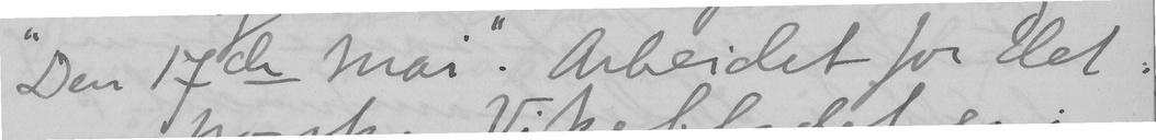"Den 17de mai". Arbeidet for det
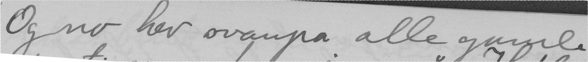Og no hev ovanpå alle gamle
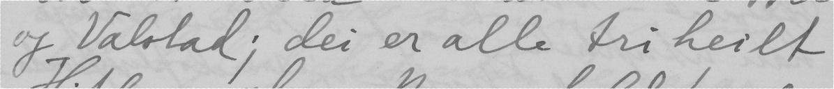og Valstad; dei er alle tri heilt
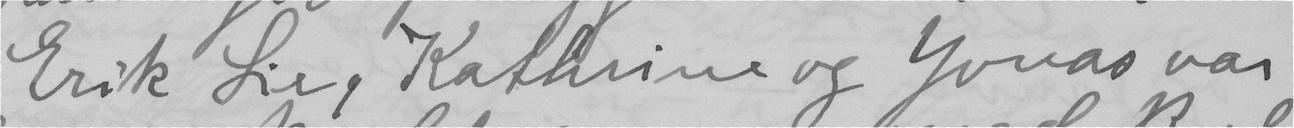Erik Lie, Kathrine og Jonas var
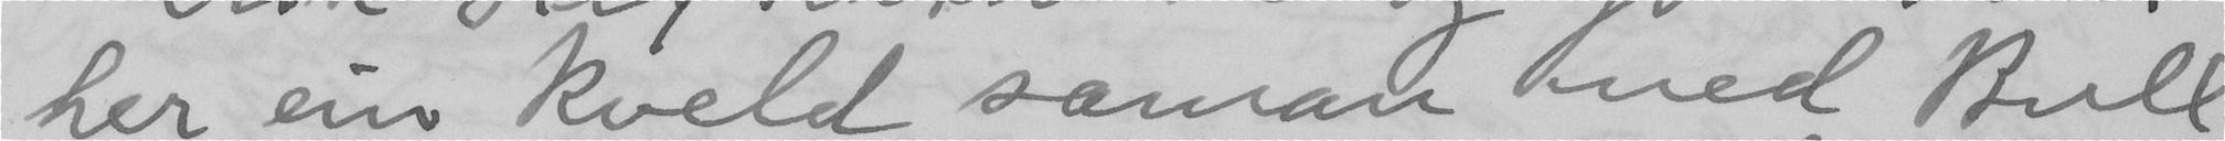her ein kveld saman med Bull
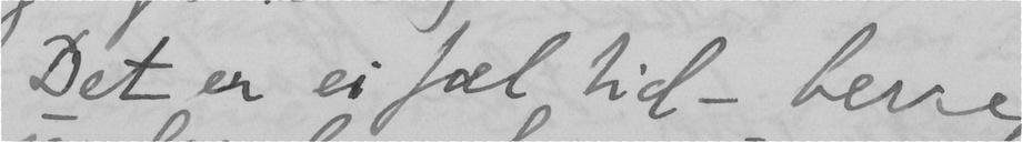Det er ei fæl tid – berre
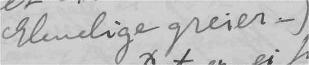Elendige greier.
